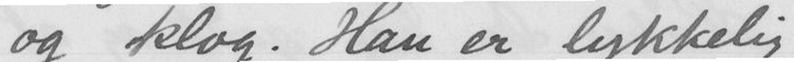og klog. Han er lykkelig
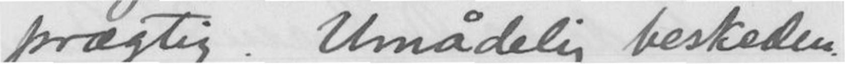prægtig. Umådelig beskeden
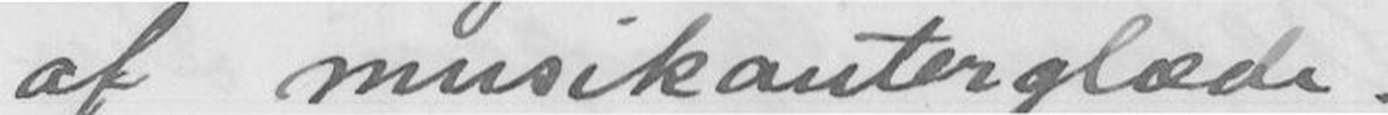af musikanterglæde.
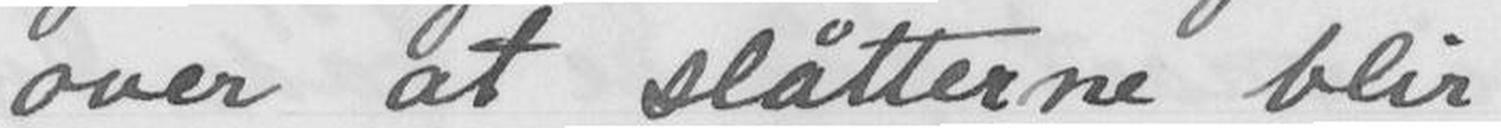over at slåtterne blir
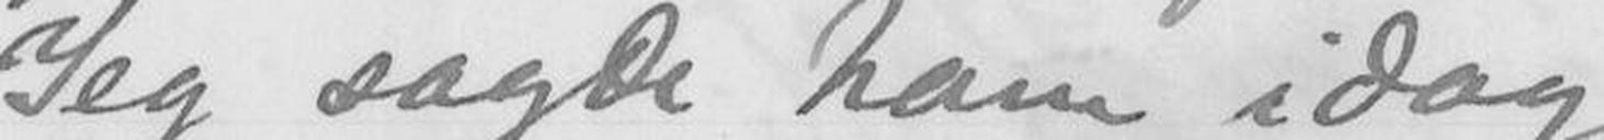Jeg sagde ham idag
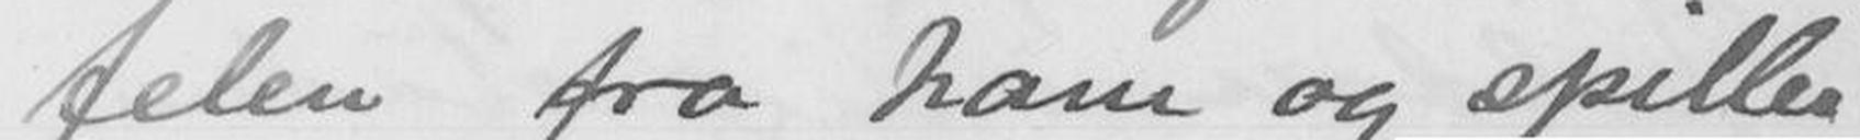felen fra ham og spiller
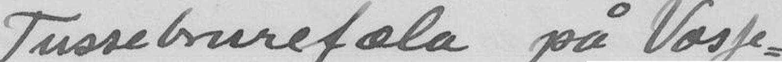Tussebrurefæla på Vosse-
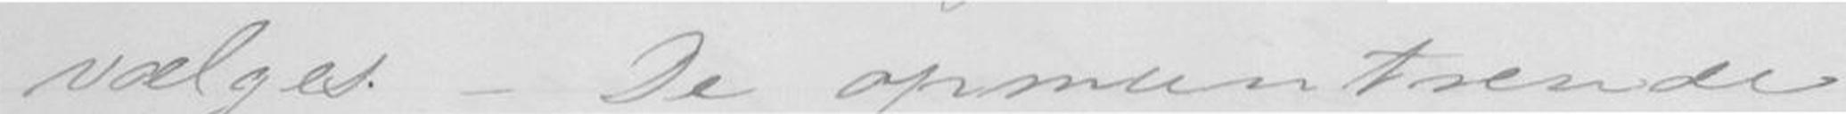vælges. - De opmuntrende
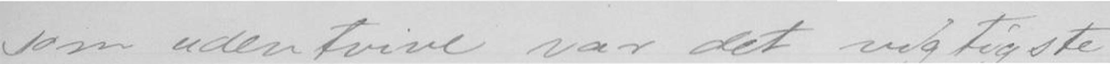som uden tvivl var det vigtigste
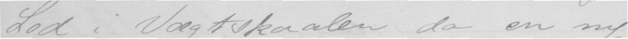Lod i Vægtskaalen da en ny
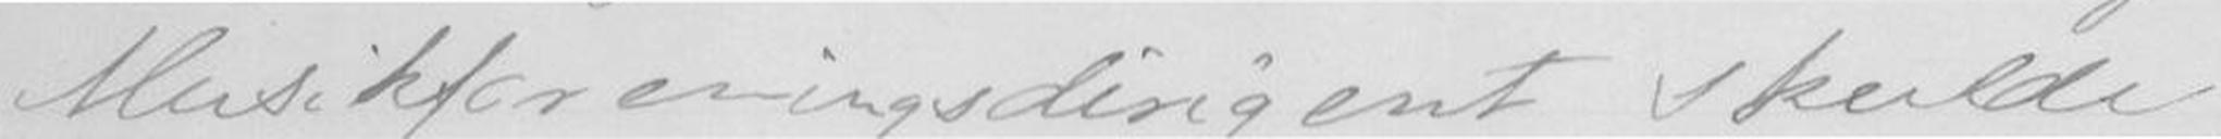Musikforeningsdirigent skulde
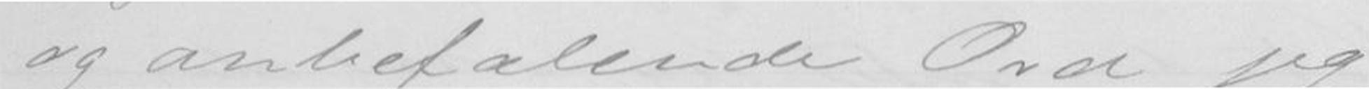og anbefalende Ord jeg
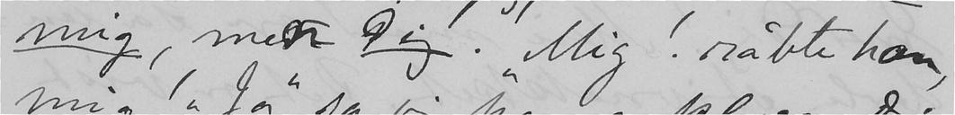mig, men Dig. "Mig! råbte han,
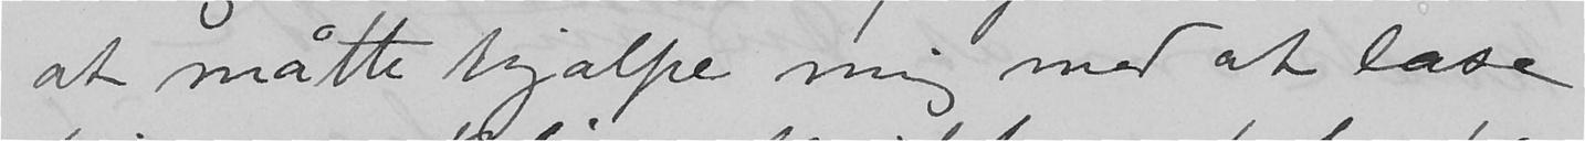at måtte hjælpe mig med at læse
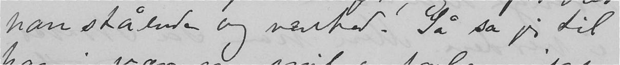han stående og vented. Så sa jeg til
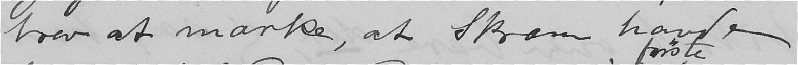brev at mærke, at Skram havde
